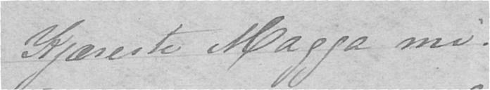Kjæreste Magga mi.
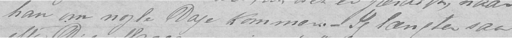han om nogle Dage kommer. Jeg længter saa
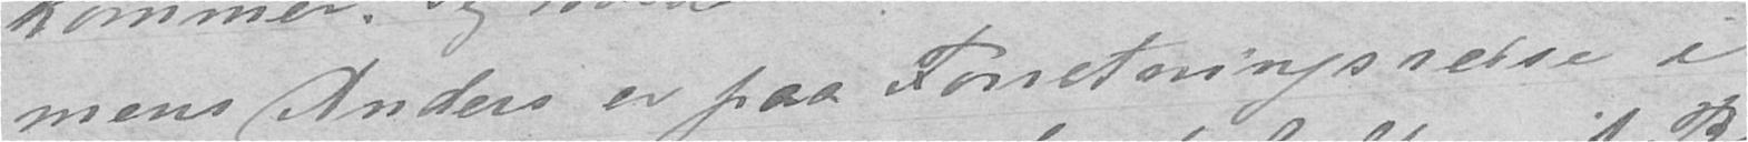mens Anders er paa Forretningsreise i
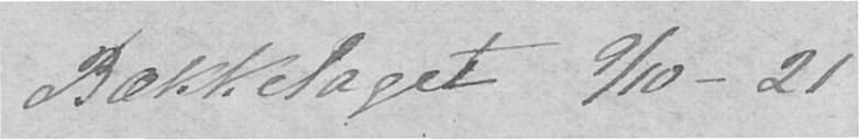Bækkelaget 9/10- 21
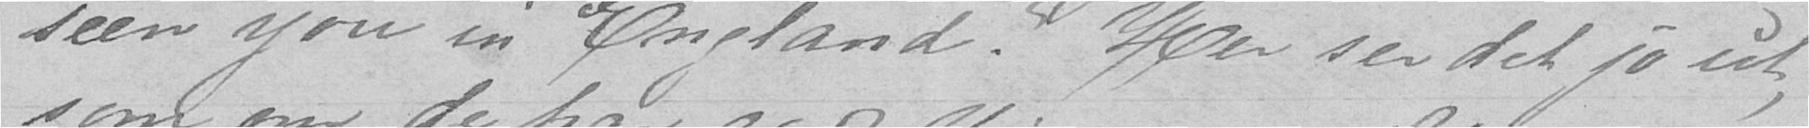seen you in England. Her ser det jo ut,
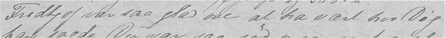Fridtjof var saa glad over at ha vært hos Dig
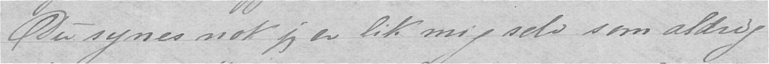Du synes nok jeg er lik mig selv som aldrig
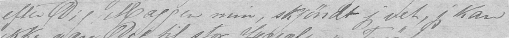efter Dig, Maggen min, skjøndt jeg vet, jeg kan
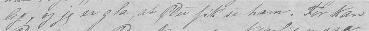lig, og jeg er gla, at Du fik se ham. For kan
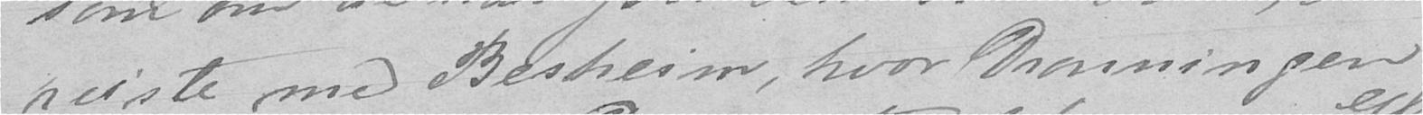reiste med Besheim, hvor Dronningen
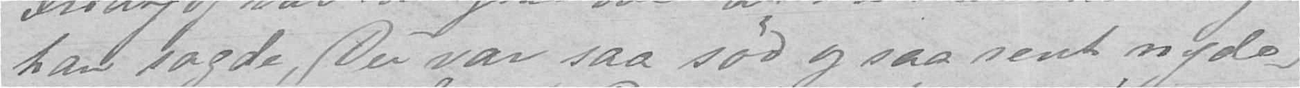han sagde, Du var saa sød og saa rent nyde-
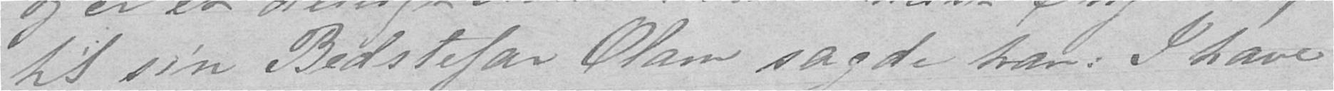til sin Bedstefar Olam sagde han: I have
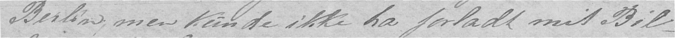Berlin, men kunde ikke ha forladt mit Bil-
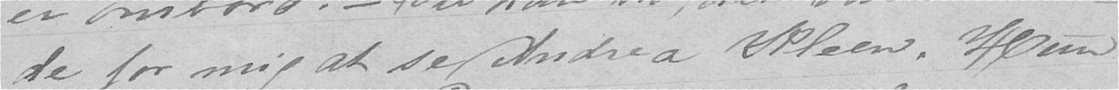de for mig at se Andrea Kleen. Hun
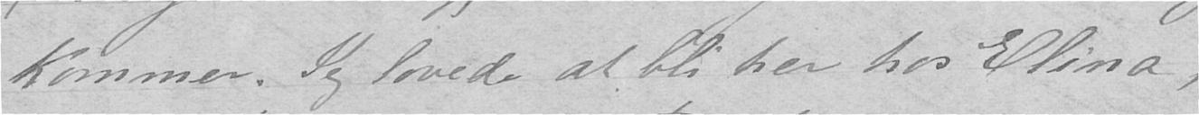kommer. Jeg lovede at bli her hos Elina,
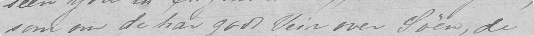som om de har godt Veir over Søen, de
